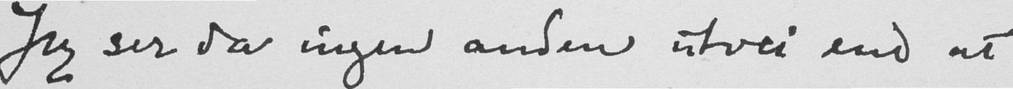Jeg ser da ingen anden utvei end at
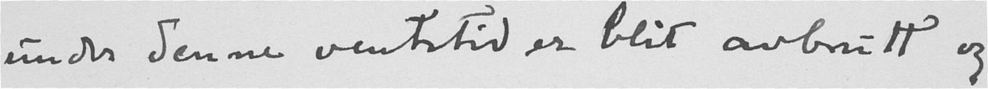under denne ventetid er blit avbrutt og
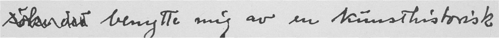søke det benytte mig av en kunsthistorisk
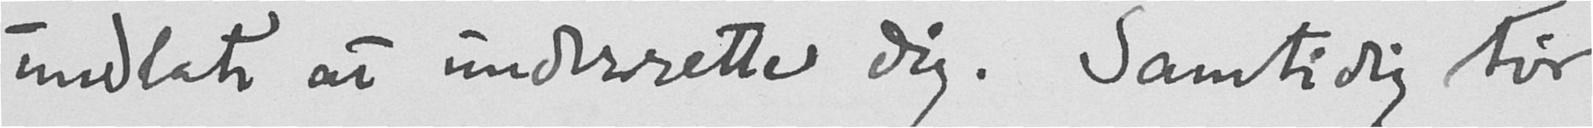undlate at underrette dig. Samtidig tør
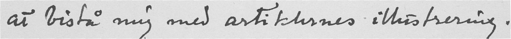at bistå mig med artiklernes illustrering.
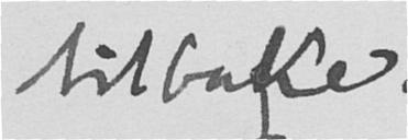tilbake.
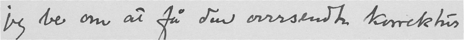jeg be om at få den oversendte korrektur
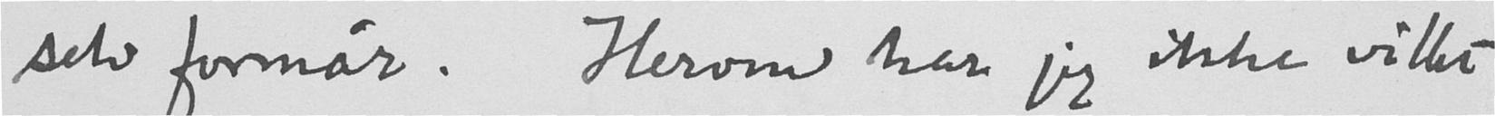selv formår. Herom har jeg ikke villet
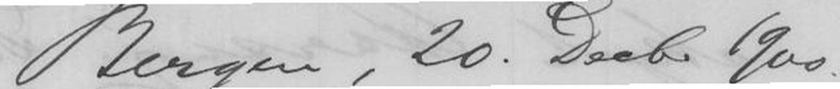Bergen, 20 Decb 1900.
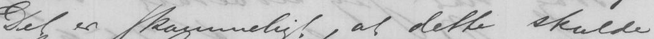Det er skammeligt, at dette skulde
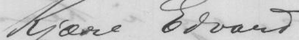Kjære Edvard!
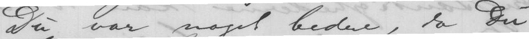Du var noget bedre, da Du
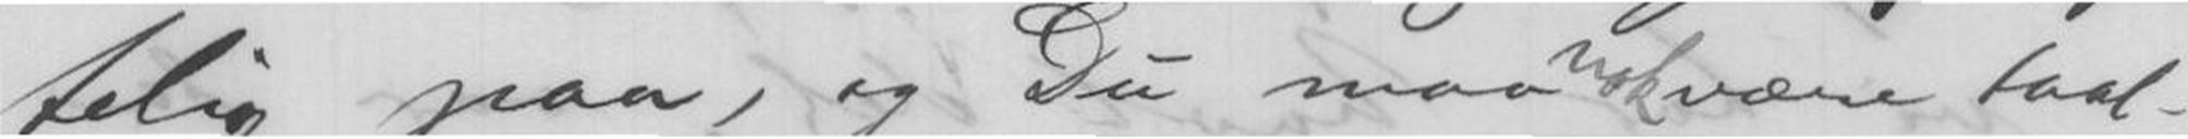telig paa, og Du maa nok være taal-
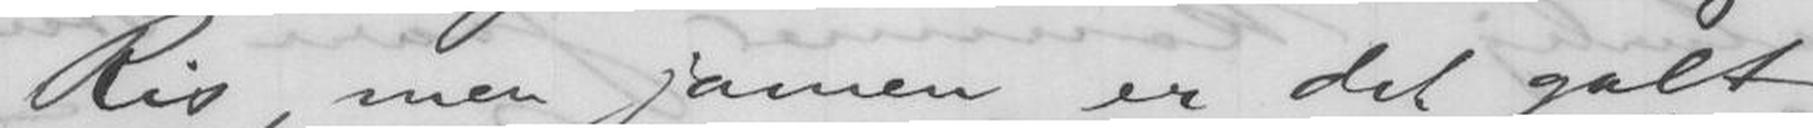Ris, men jammen er det galt
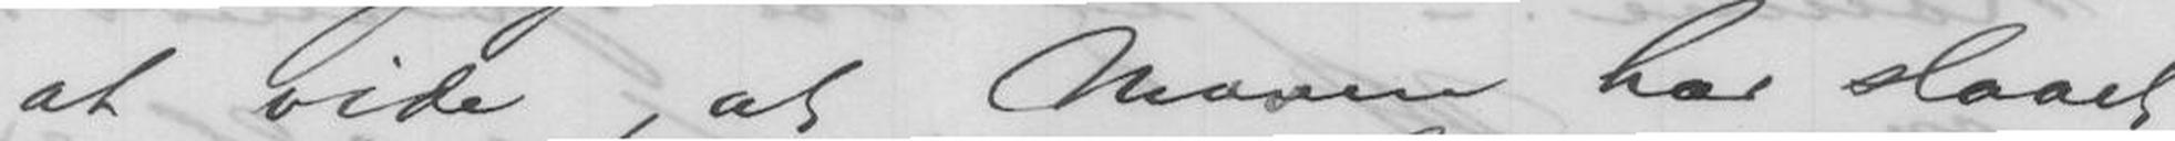at vide, at Maven har slaaet
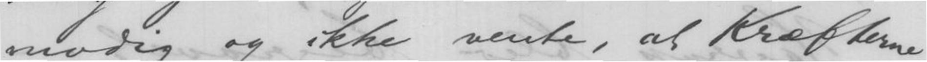modig og ikke vente, at Kræfterne
